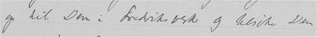op til Dem i Fredriksverk og besøke Dem
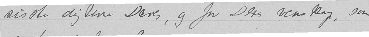sisste digtene Deres, og for Deres venskap, som
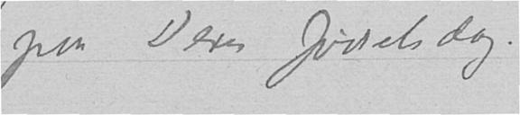paa Deres fødselsdag.
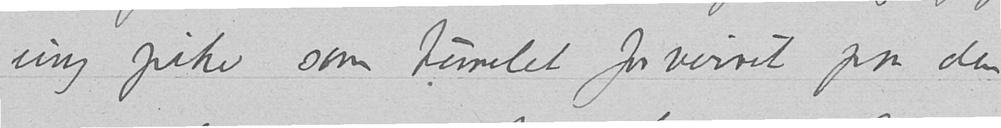ung pike som tumlet forvirret paa den
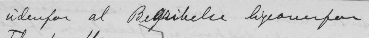udenfor al Begribelse ligeoverfor
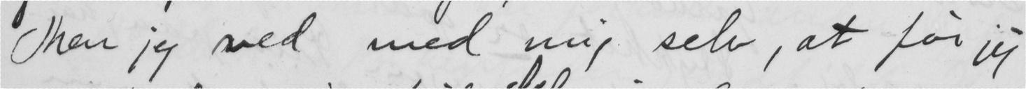Men jeg ved med mig selv, at før jeg
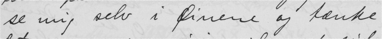se mig selv i Øinene og tænke
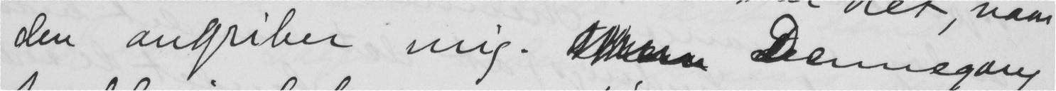den angriber mig.  Dennegang
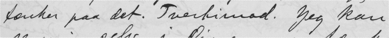tænker paa det. Tvertimod. Jeg kan
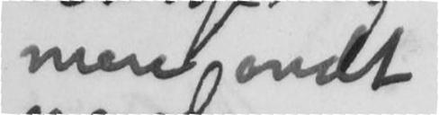mere ondt
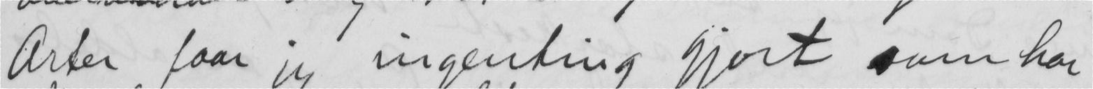Arter faar jeg ingenting gjort som har
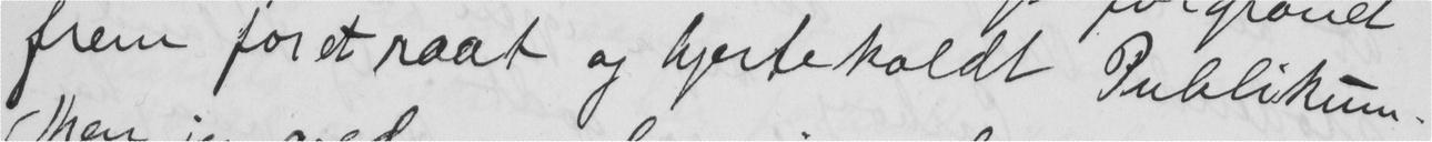frem føret raat og hjertekoldt Publikum.
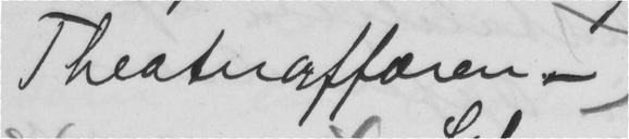Theateraffæren -
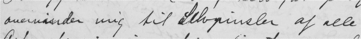overvinder mig til Selvpinsler af alle
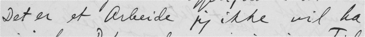Det er et Arbeide jeg ikke vil ha
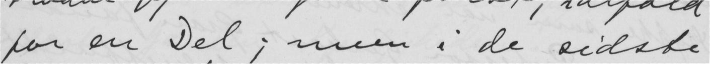for en Del; men i de sidste
